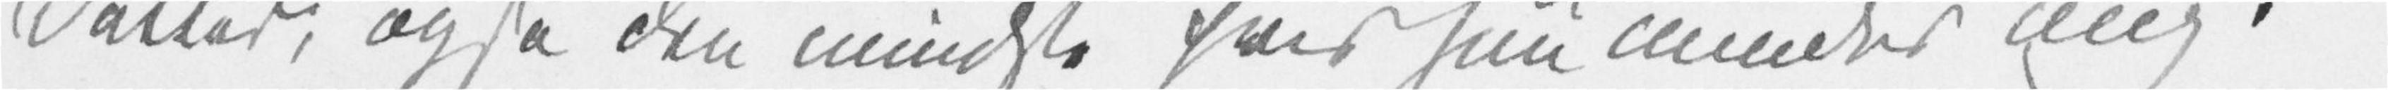Datter, også den mindste hvis hun mindes mig.
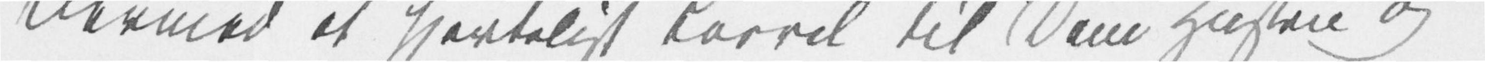Dermed et hjerteligt Levvel til Dem Hustru og
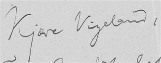Kjære Vigeland,
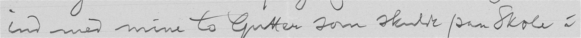ind med mine to Gutter som skulde paa Skole i
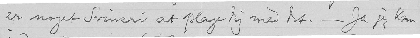er noget Svineri at plage dig med det. - Ja jeg kom
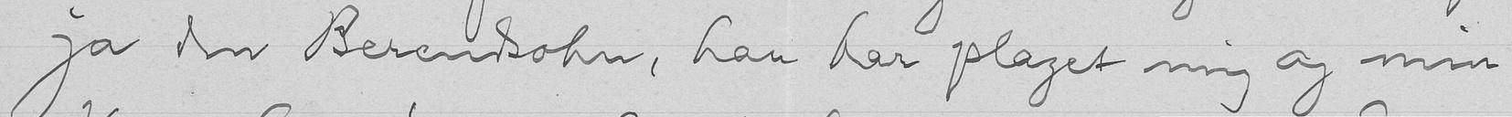ja den Berendsohn, han har plaget mig og min
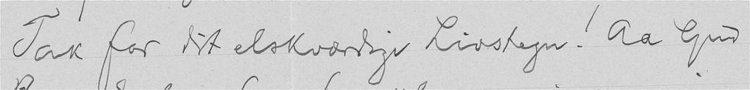Tak for dit elskværdige Livstegn! Aa Gud
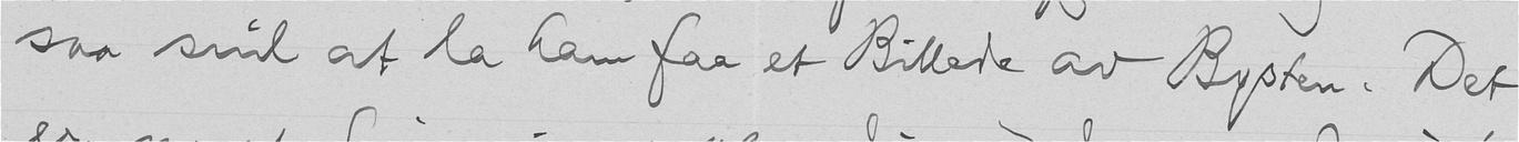saa snil at la ham faa et Billede av Bysten. Det
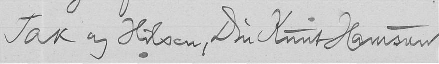Tak og Hilsen, Din Knut Hamsun
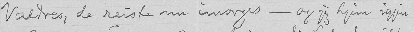Valdres, de reiste nu imorges - og jeg hjem igjen
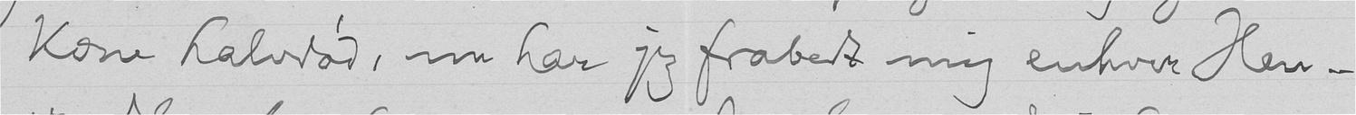Kone halvdød, nu har jeg frabedt mig enhver Hen-
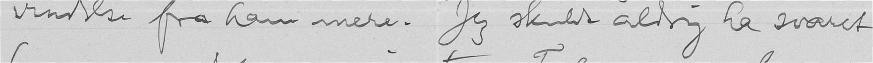vendelse fra ham mere. Jeg skulde aldrig ha svaret
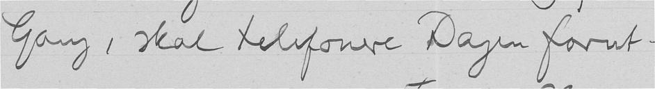Gang, skal telefonere Dagen forut.
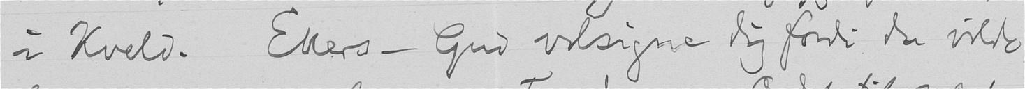i Kveld. Ellers - Gud velsigne dig fordi du vilde
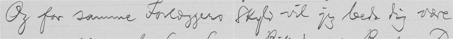Og for samme Forlæggers Skyld vil jeg bede dig være
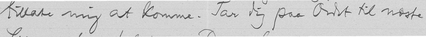tillate mig at komme. Tar dig paa Ordet til næste
